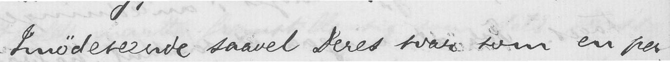Imødeseende saavel Deres svar som en per-
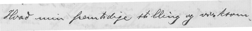Hvad min fremtidige stilling og virksom-
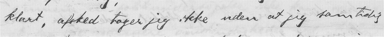klart, afsked tager jeg ikke uden at jeg samtidig
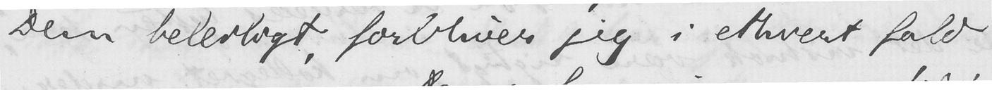Dem beleiligt, forbliver jeg i ethvert fald
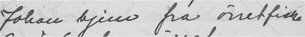Johan hjem fra ørretfiske
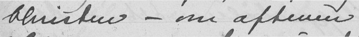Christen - om aftenen
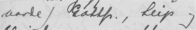vaade) Gottfr., Seip og
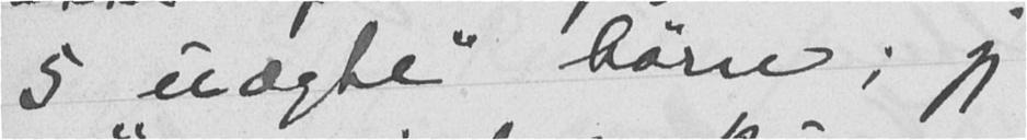5 "uægte" børn; jeg
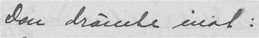Don drømte inat:
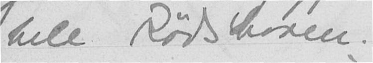hele Rødshaven.
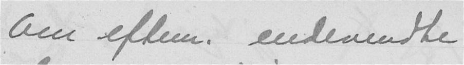Om efterm. endevendte
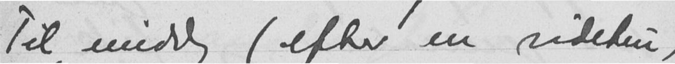Til middag (efter en ridetur,
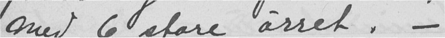med 6 store ørret. -
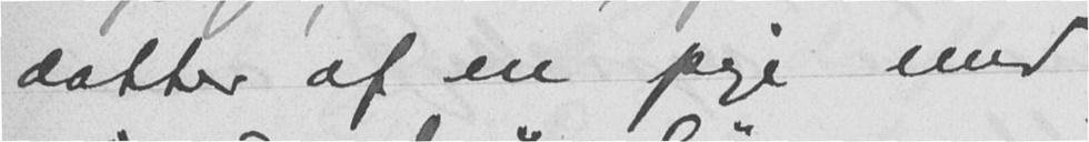datter af en pige med
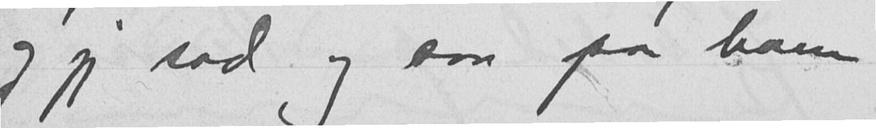og jeg sad og saa paa ham
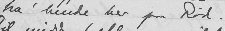ha' hende her paa Rød.
